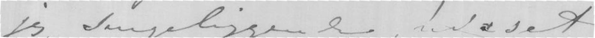jeg sengeliggende, udaset,
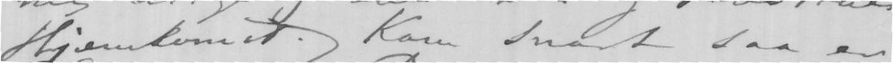Hjemkomst. Kom snart saa er
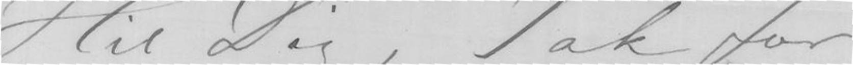Hil Dig, Tak for
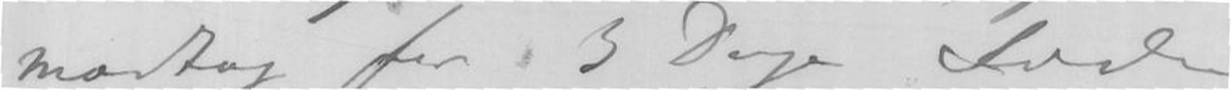modtog for 3 Dage Siden,
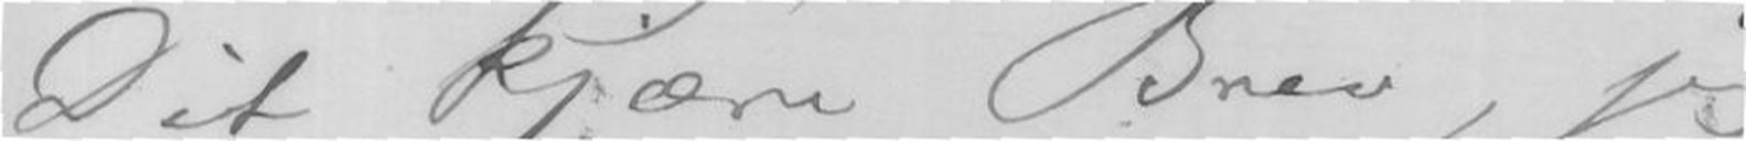Dit kjære Brev, jeg
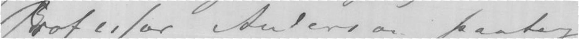Professor Anderson paatog
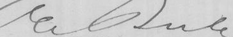Ole Bull
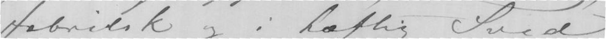febrilsk og i hæftig Sved
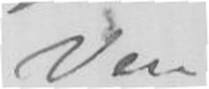Ven
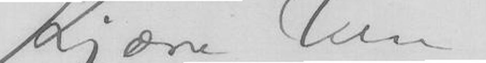Kjære Ven,
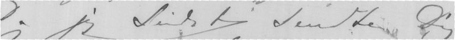Da jeg sidst Sendte Dig
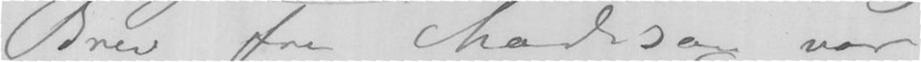Brev fra Madison var
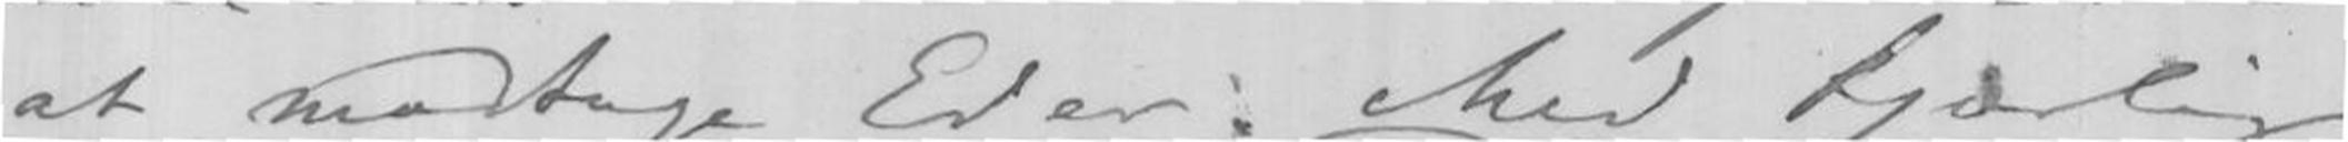at modtage Eder. Med kjærlig
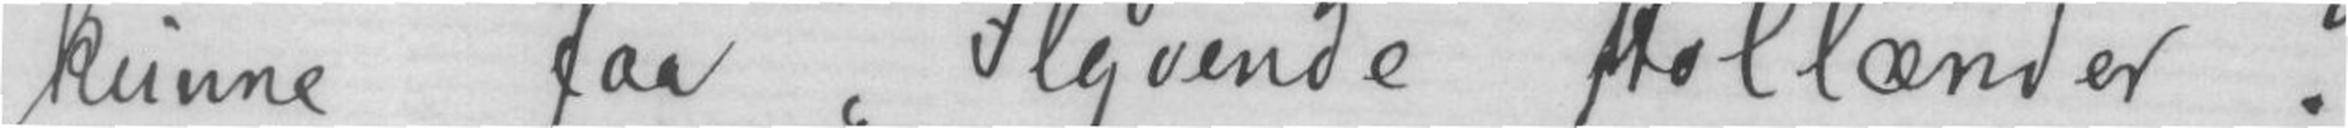kunne faa Flyvende Hollænder?
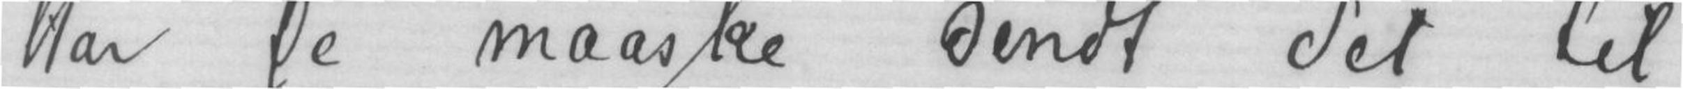Har De maaske sendt det til
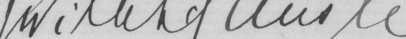Wilhelm Hansen
